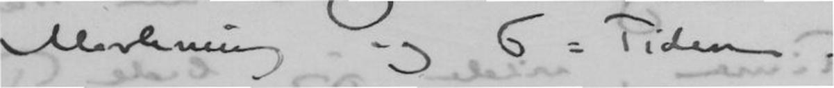Mørkning og 6 = Tiden.
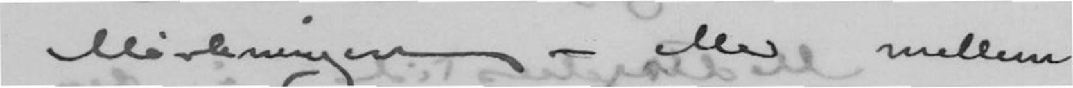i Mørkningen - Men mellem
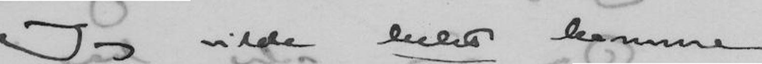Jeg vilde helst komme
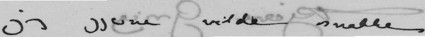jeg gjerne vilde snakke
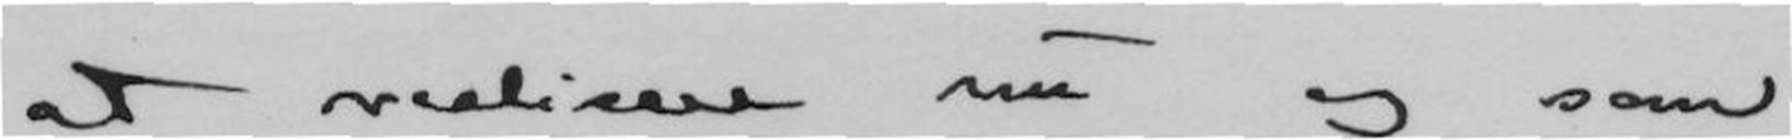at realisere nu og som
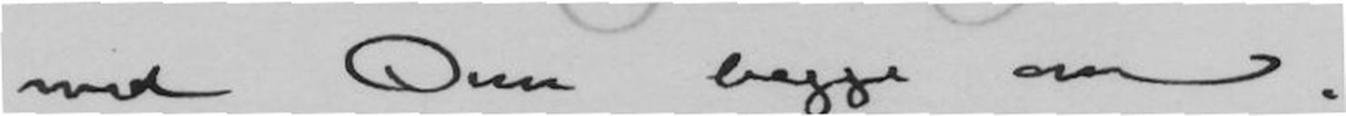med Dem begge om.
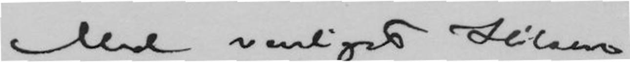Med venligst Hilsen
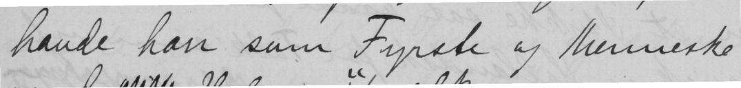havde han som Fyrste og Menneske
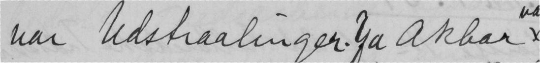var Udstraalinger. Ja Akbar
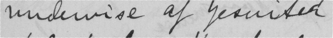undervise af jesuiter
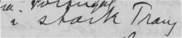i stærk Trang
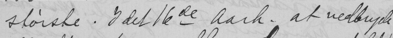største. I det 16de Aarh. at nedbryde
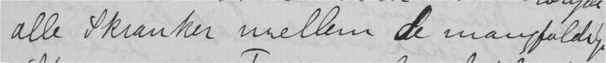alle Skranker mellem de mangfoldige
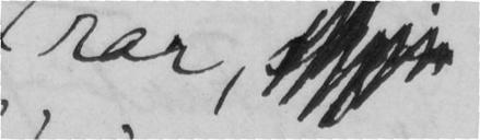rar,
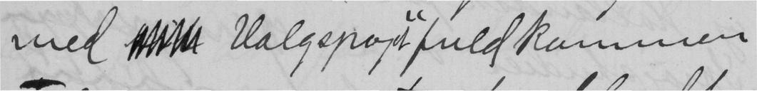med  Valgsprog "fuldkommen
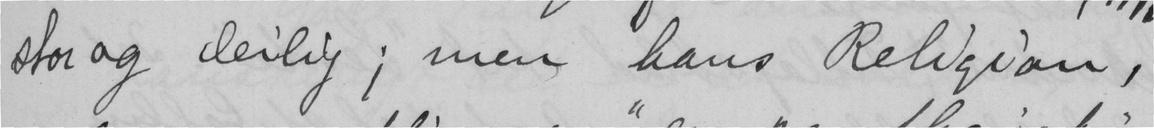stor og deilig; men hans Religion,
